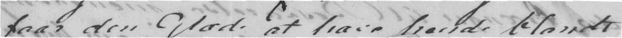faar den Glæde at have hende blandt
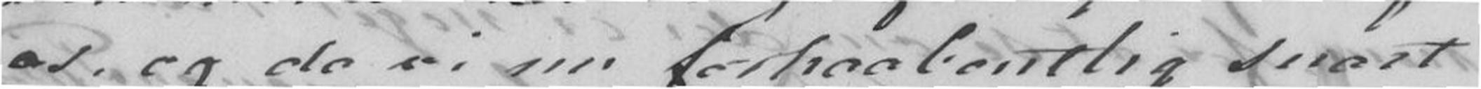os, og da vi nu forhaabentlig snart
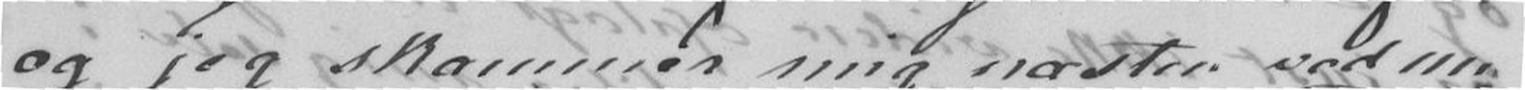og jeg skammer mig næsten ved nu
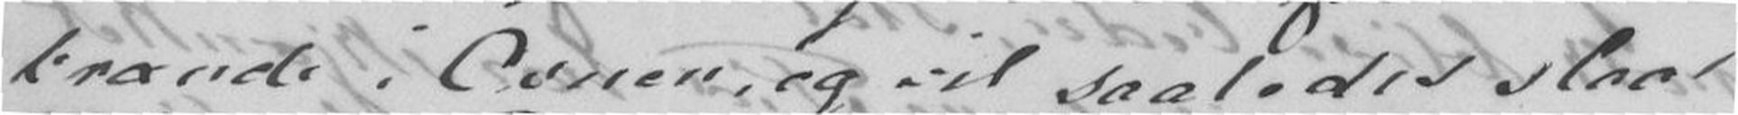brænde i Ovnen, og vil saaledes slaa
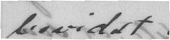bevidst
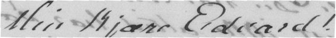Min kjære Edvard!
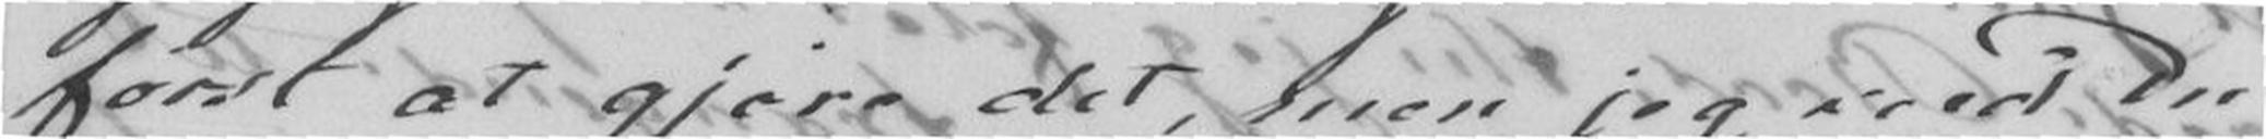først at gjøre det, men jeg veed Du
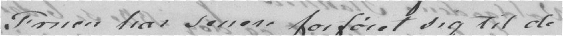Fruen har senere forføiet sig til de
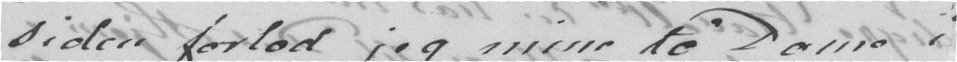siden forlod jeg mine to Dame i
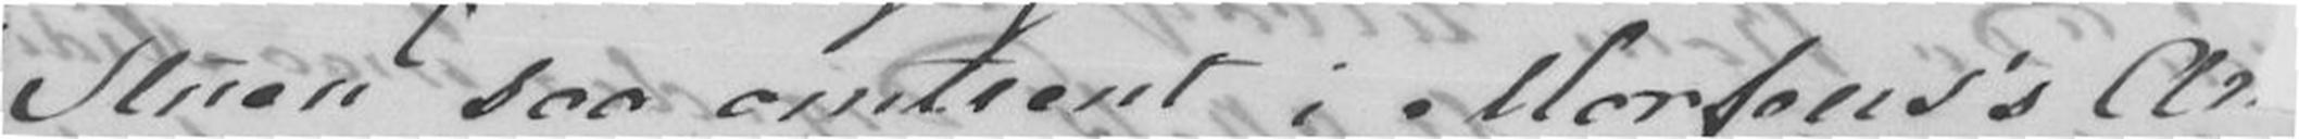Stuen saa omtrent i Morfaers's Arme.
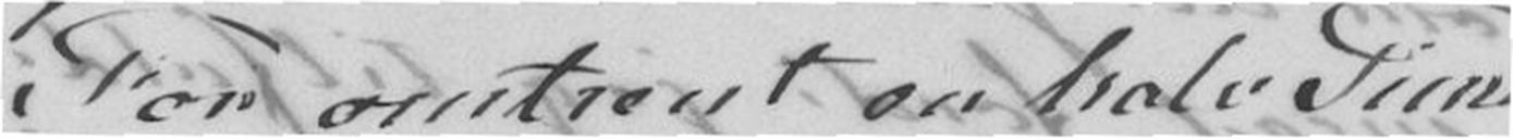For omtrent en halv Time
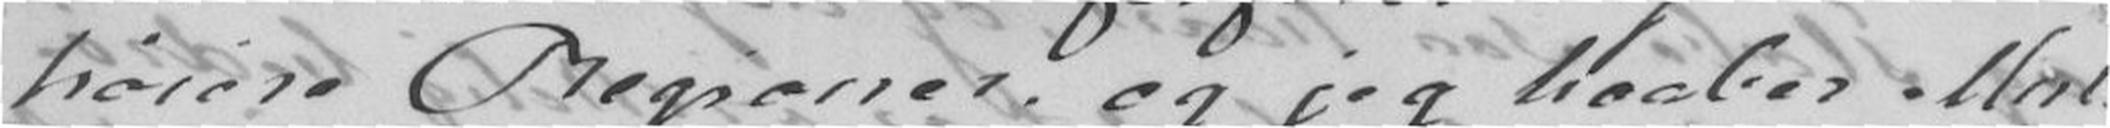høiere Regioner og jeg haaber Mut-
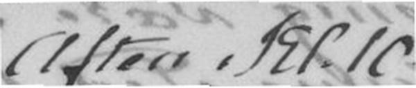Aften Kl.10
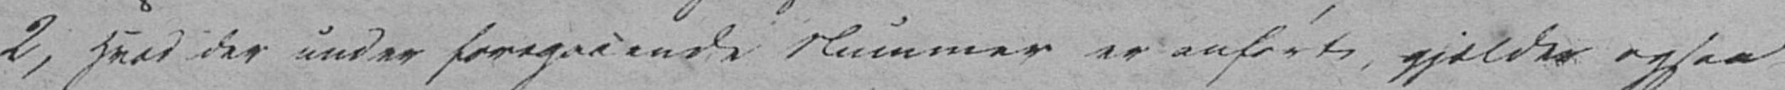2, Hvad der under foregaaende Nummer er anført, gjælder ogsaa
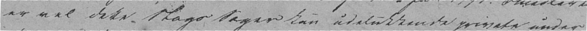er vel dette Slags Sager kun udelukkende private under
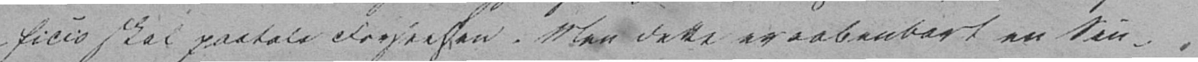ficio skal paatale Forseelsen. Men dette er aabenbart en Sin-
